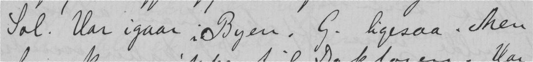Sol. Var igaar i Byen. G. ligesaa. Men
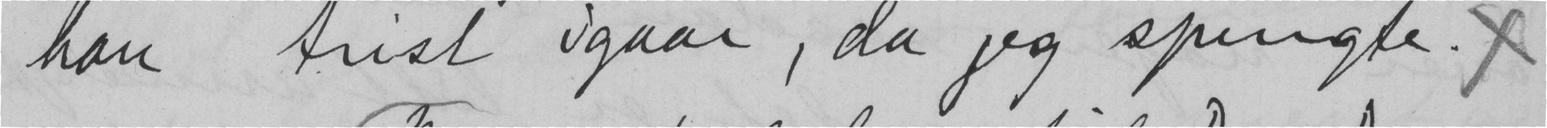han trist igaar, da jeg spurgte. x
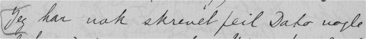Jeg har nok skrevet feil Dato nogle
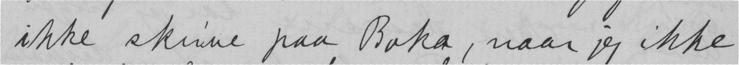ikke skrive paa Boka, naar jeg ikke
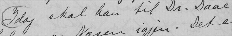Idag skal han til Dr. Daae
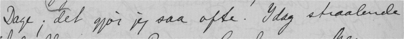Dage; det gjør jeg saa ofte. Idag straalende
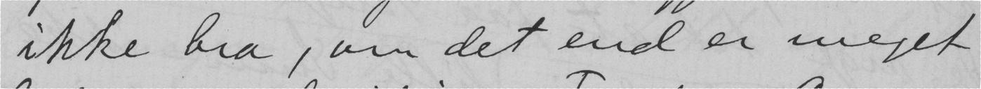ikke bra, om det end er meget
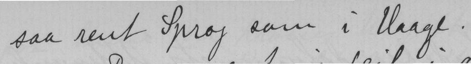saa rent Sprog som i Vaage.
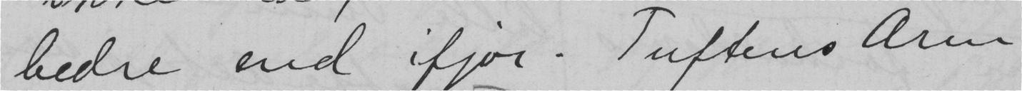bedre end ifjor. Tuftens arm
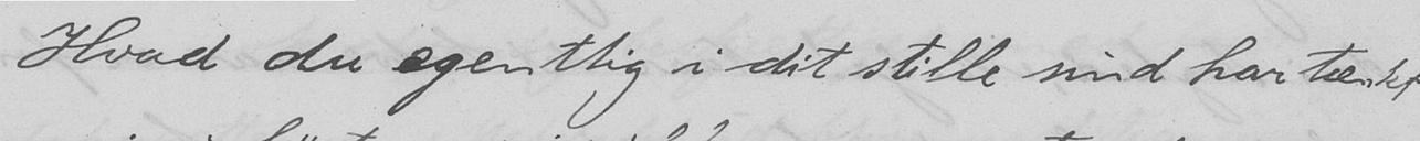Hvad du egentlig i dit stille sind har tænkt
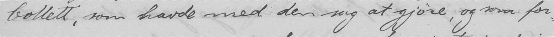Collett, som havde med den sag at gjøre, og som for-
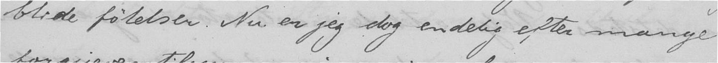blide følelser. Nu er jeg dog endelig efter mange
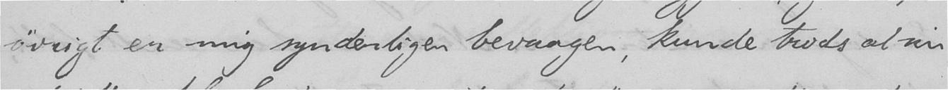øvrigt er mig synderligen bevaagen, kunde trods al sin
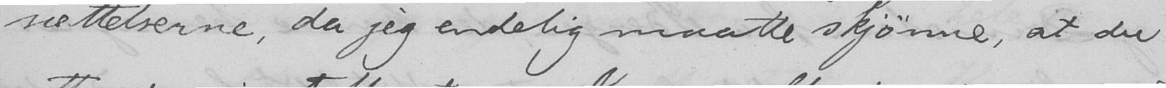rettelserne, da jeg endelig maatte skjønne, at du
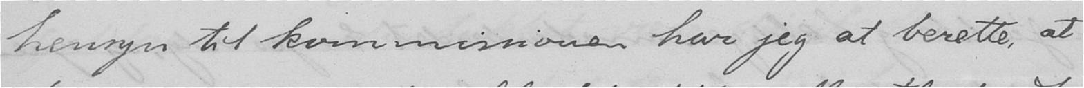hensyn til kommissionen har jeg at berette, at
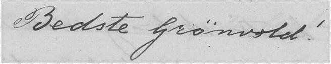Bedste Grønvold!
